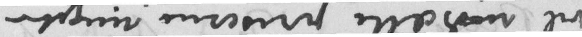vil nedsætte priserne meget
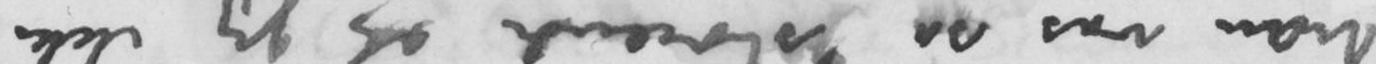han var så støiende at jeg ikke
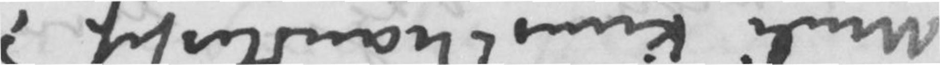Muli Kunsthandlerfif?
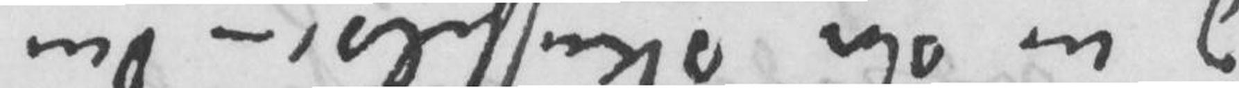og en stor skuffelse – Den
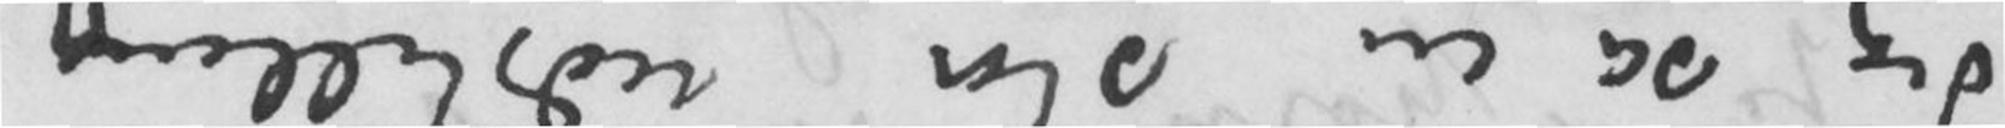Jeg så en stor udstilling
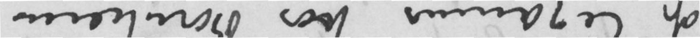af Cezannes hos Bernheim
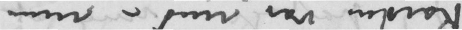Karsten var med – men
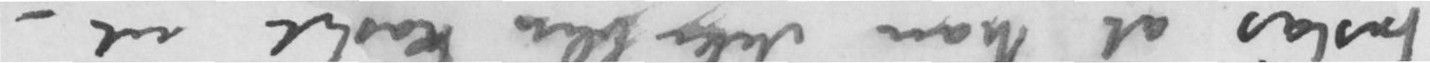forstår at han ikke blev kastet ut –
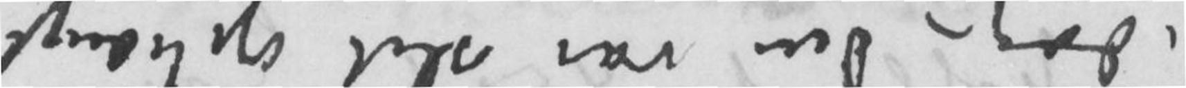idag – Den var slet ophængt
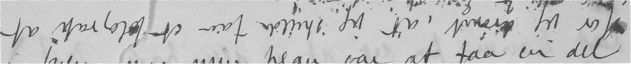Har jeg drømt, at jeg skulde faa et fotografi af
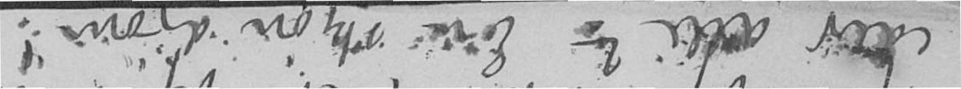eder alle? En skjøn drøm!
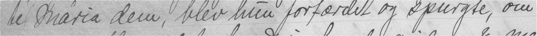te Maria dem, blev hun forfærdet og spurgte, om
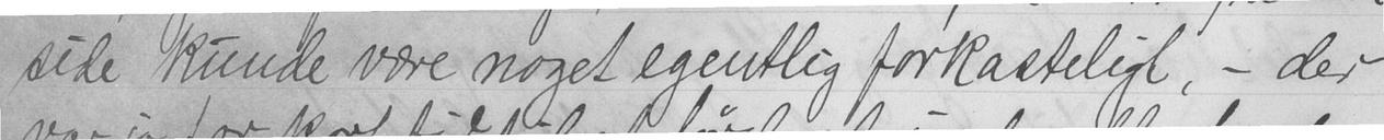side kunde være noget egentlig forkasteligt, - der
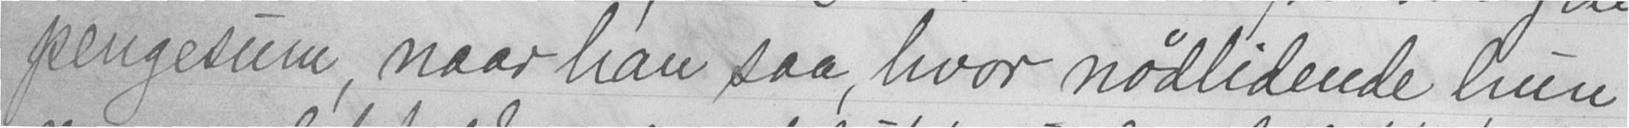pengesum, naar han saa, hvor nødlidende hun
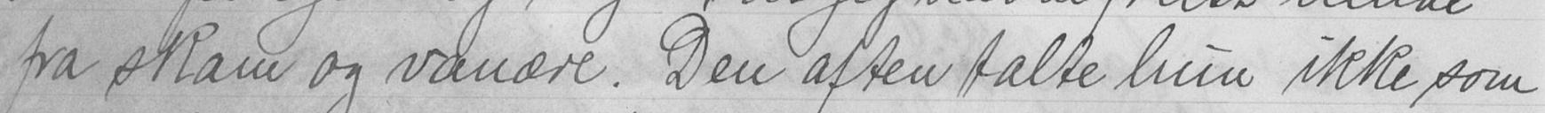fra skam og vanære. Den aften talte hun ikke som
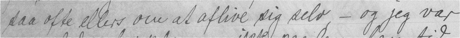saa ofte ellers om at aflive sig selv, - og jeg var
